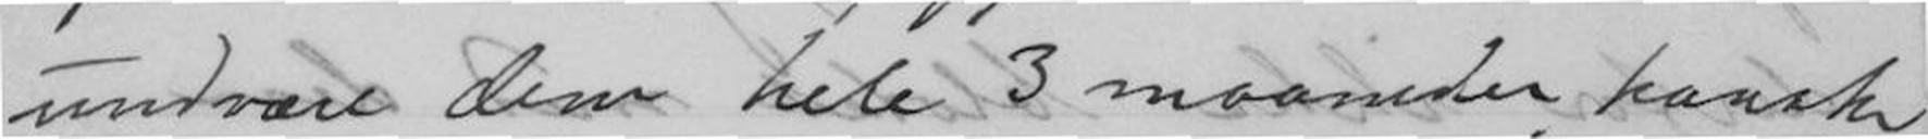at undvære dem hele 3 maaneder kanske
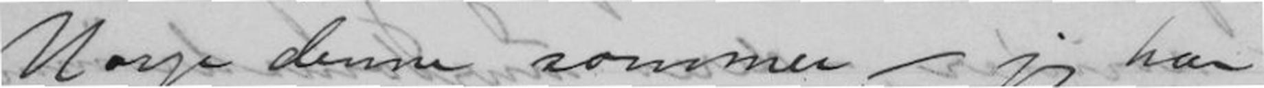Norge denne sommer, - jeg har
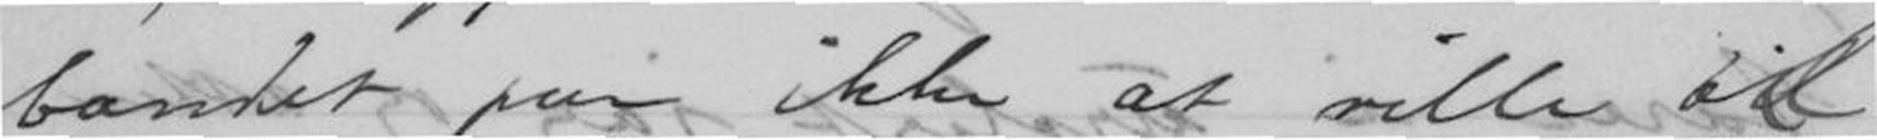bandet paa ikke at ville til
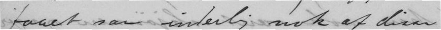faaet saa inderlig nok af disse
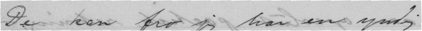De kan tro jeg har en yndig
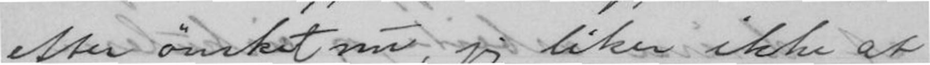efter ønsket nu, jeg liker ikke
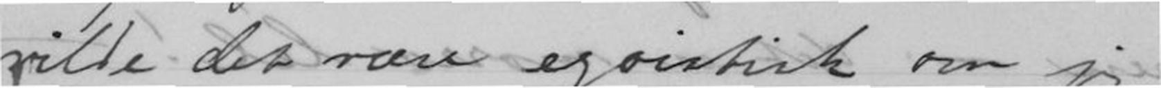vilde det være egoistisk om jeg
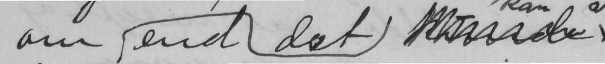om end det
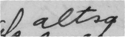altsa
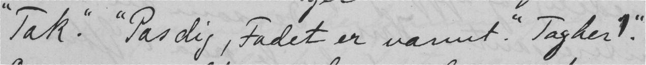"Tak". "Pas dig, Fadet er varmt". "Tag her!".
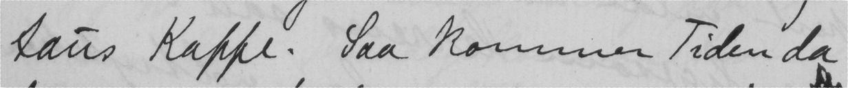taus Kaffe. Saa kommer Tiden da
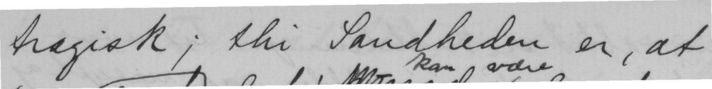tragisk; thi Sandheden er, at
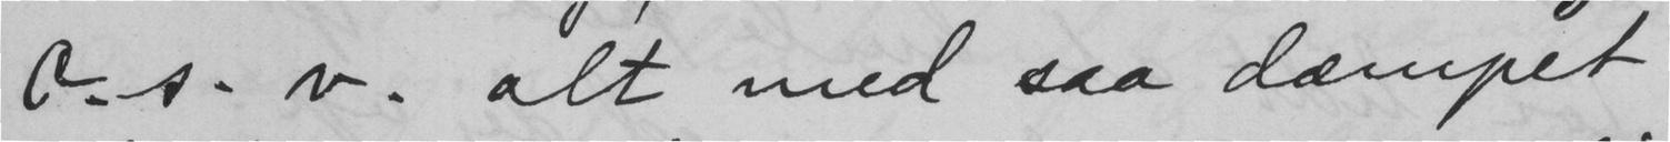o.s. v. alt med saa dæmpet
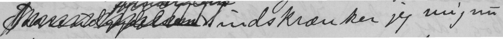indskrænker jeg mig nu
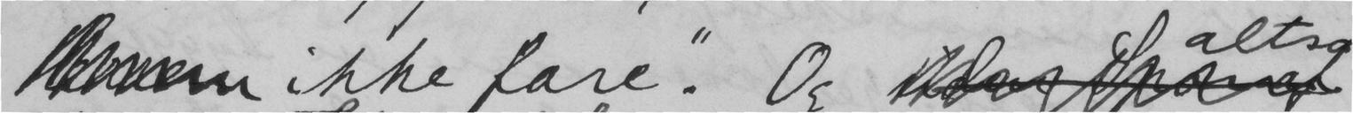ikke fare". Og
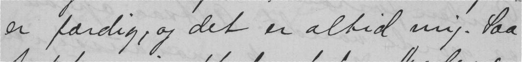er færdig, og det er altid mig. Saa
